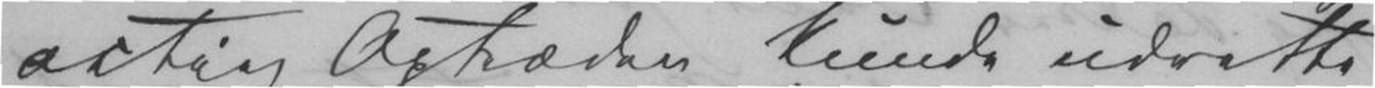activ Optræden kunde udrette
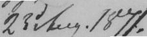23 Aug. 1871.
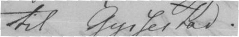til Gyssestad.
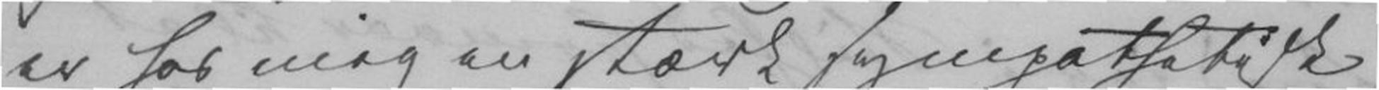er for mig en stærk sympathietisk
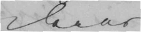Deres
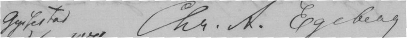Gyssestad Chr.A. Egebert
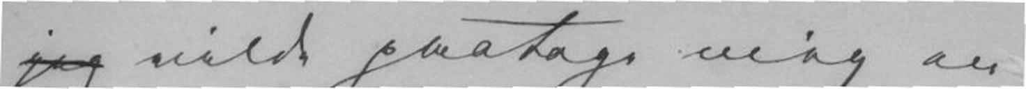jeg vilde paatage mig en
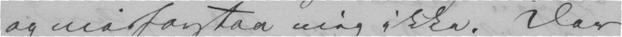og misforstaa mig ikke. Der
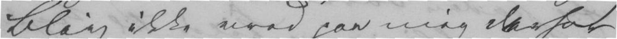Bliv ikke vred paa mig derfor
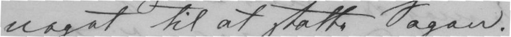noget til at støtte Sagen.
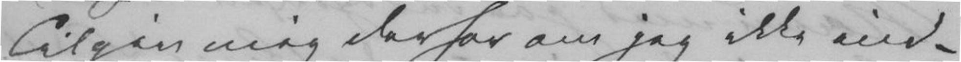Tilgiv mig derfor om jeg ikke ind-
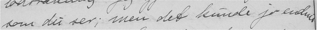som du ser; men det kunde jo endnu
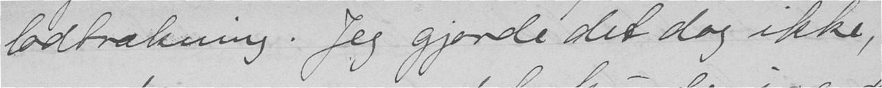lodtrækning. Jeg gjorde det dog ikke,
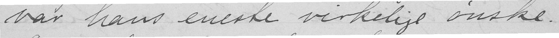var hans eneste virkelige ønske.
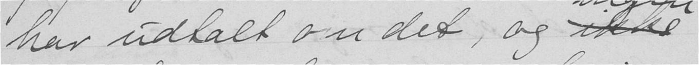har udtalt om det, og ikke
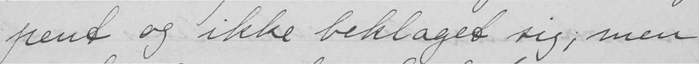pent og ikke beklaget sig; men
